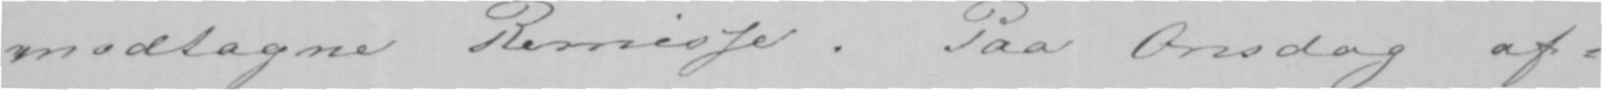modtagne Remisse. Paa Onsdag af-
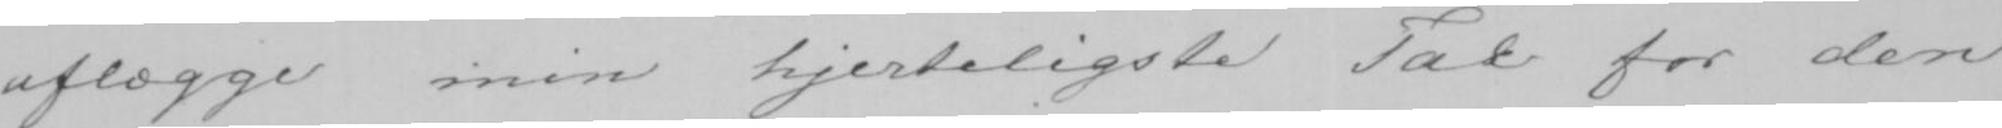aflægge min hjerteligste Tak for den
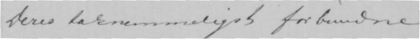Deres taknemmeligst forbundne
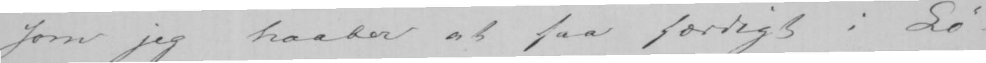som jeg haaber at faa færdigt i Lø-
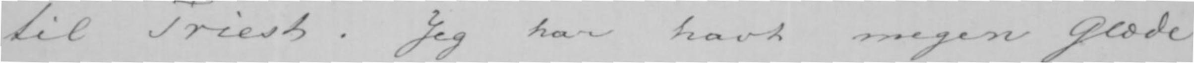til Triest. Jeg har havt megen Glæde
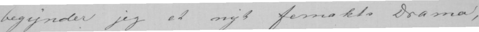begynder jeg et nyt femakts Drama,
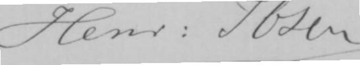Henr: Ibsen
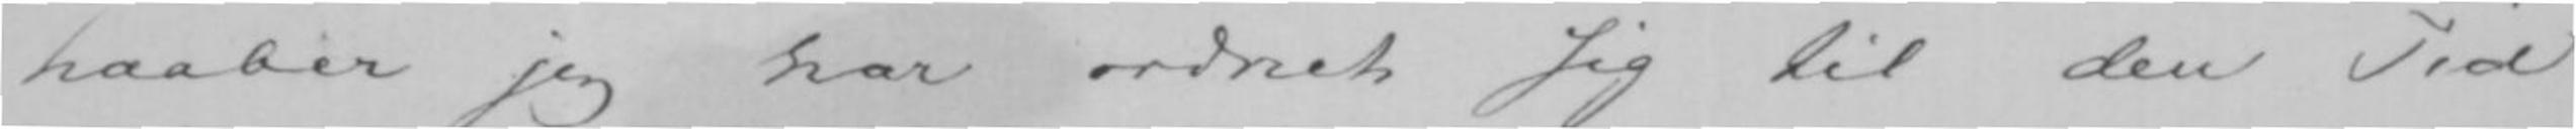haaber jeg har ordnet sig til den Tid
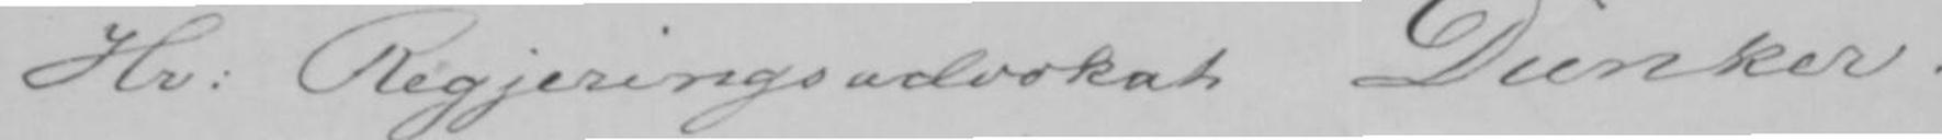Hr: Regjeringsadvokat Dunker.
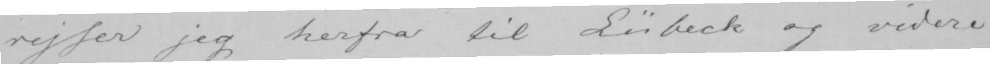rejser jeg herfra til Lübeck og videre
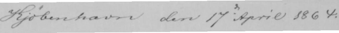Kjøbenhavn den 17de April 1864.
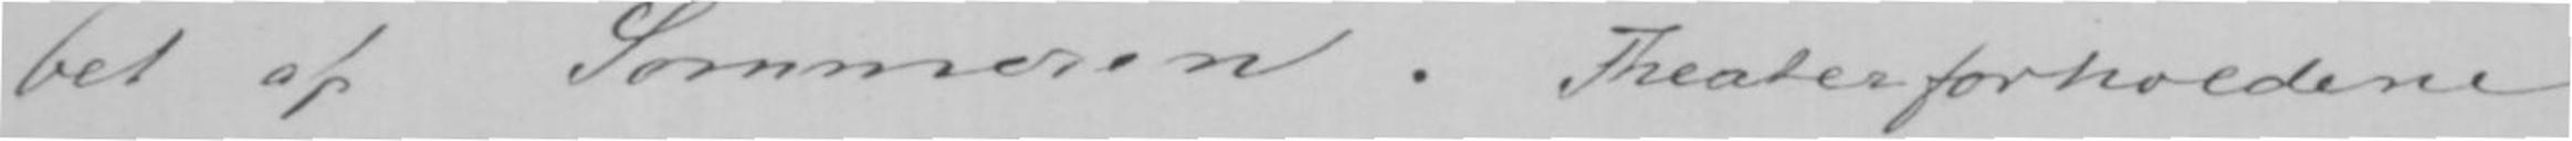bet af Sommeren. Theaterforholdene
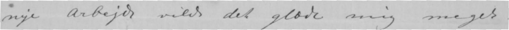nye Arbejd vilde det glæde mig meget.
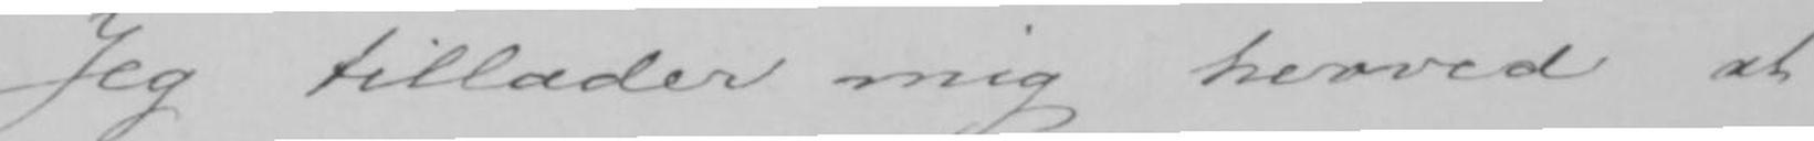Jeg tillader mig herved at
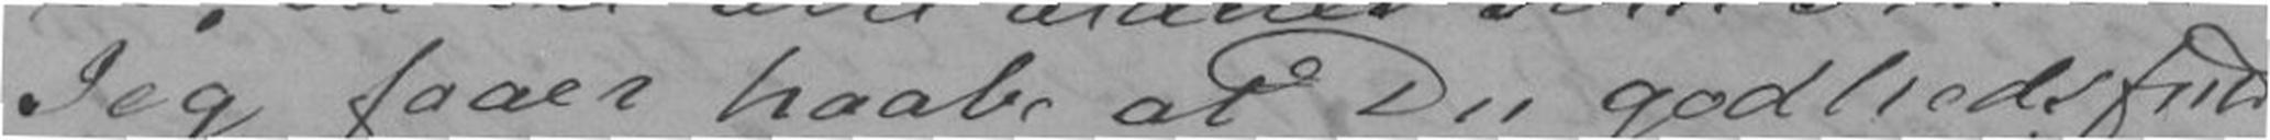Jeg faaer haabe at Du godhedsfuld
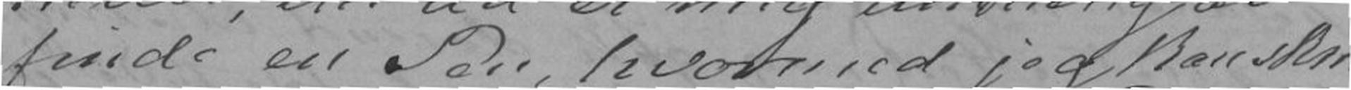finde en Pen, hvormed jeg kan skri-
